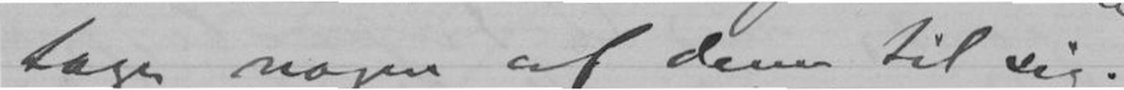tage nogen af dem til sig.
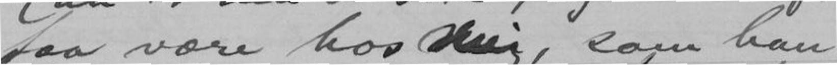faa være hos mig, som han
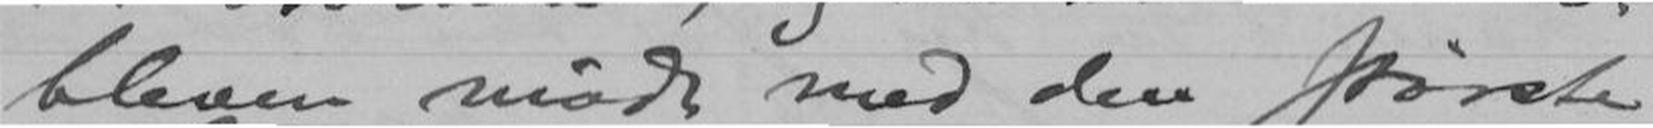bleven mødt med den største
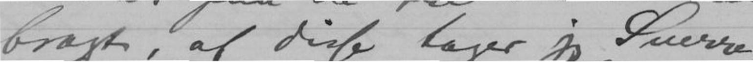bragt, af disse tager jeg Sverre.
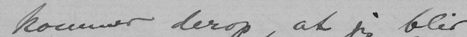jeg kommer derop, at jeg blir
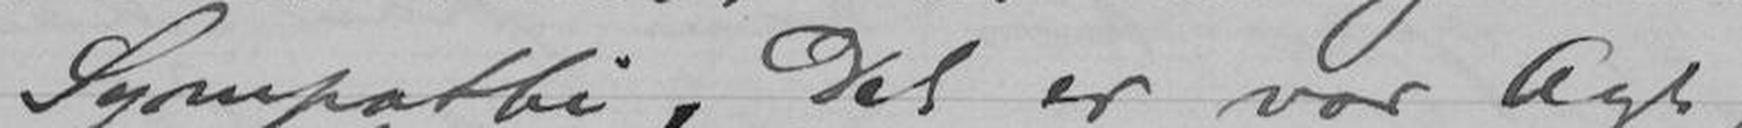Sympathi. Det er vor Agt,
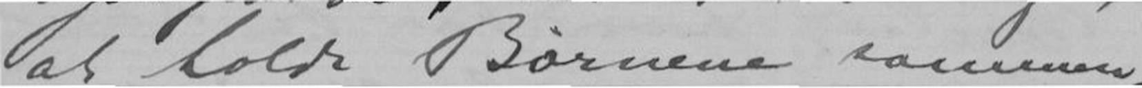at holde Børnene sammen,
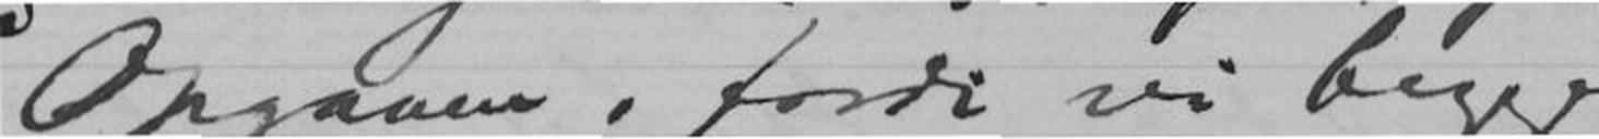Opgaven, fordi vi begge
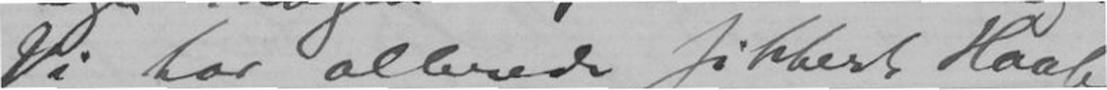Vi har allerede sikkret Haab
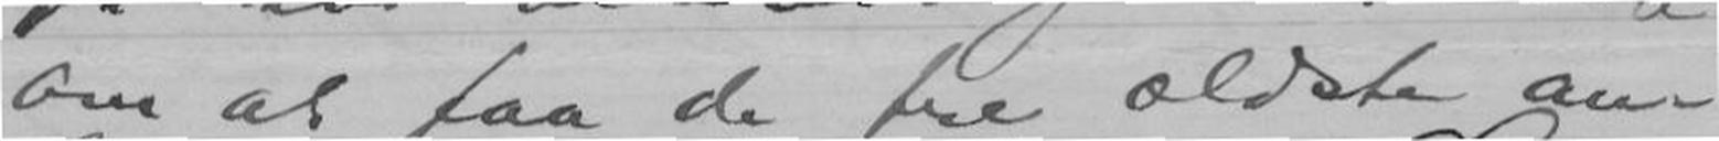om at faa de tre ældste an-
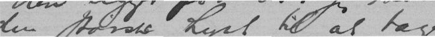den største Lyst til at tage
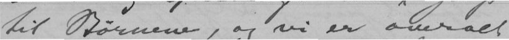til Børnene, og vi er overalt
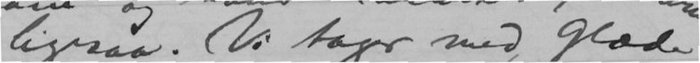ligesaa. Vi tager med Glæde
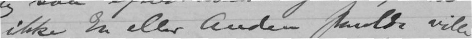ikke En eller Anden skulde ville
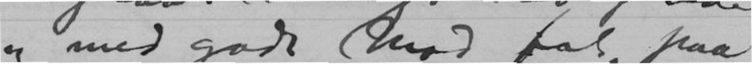og med godt Mod fat paa
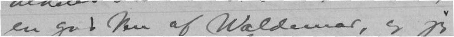en god Ven af Waldemar, og jeg
